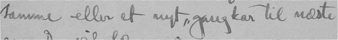samme eller et nyt "gangkar til næste
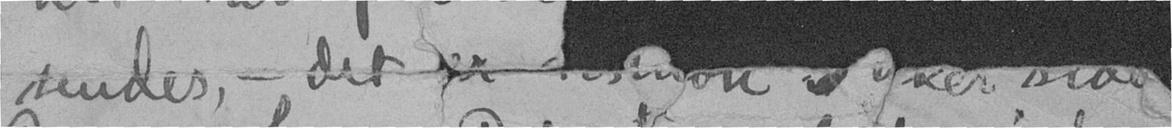sendes, - det er
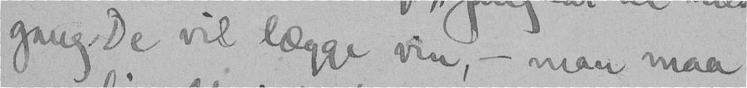gang De vil lægge vin, - man maa
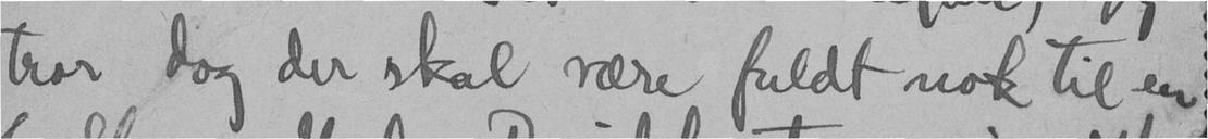tror dog der skal være fuldt nok til en
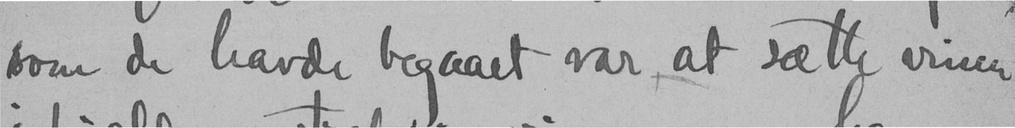som de havde begaaet var, at satte vinen
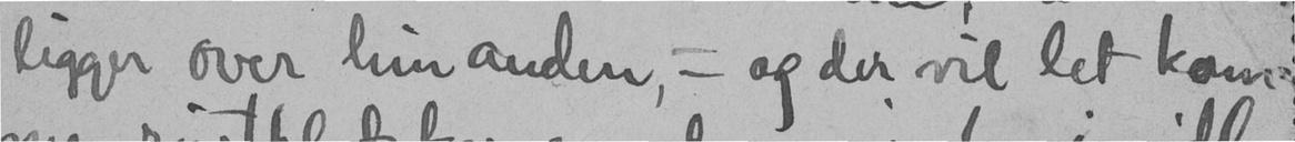ligger over hin anden, - og der vil let kom-
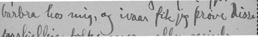barbra hos mig, og ivaar fik jeg prøve disse
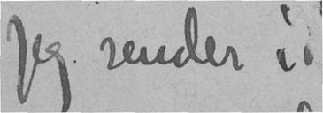Jeg sender i
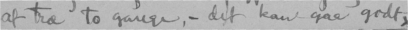af træ to gange, - det kan gaa godt;
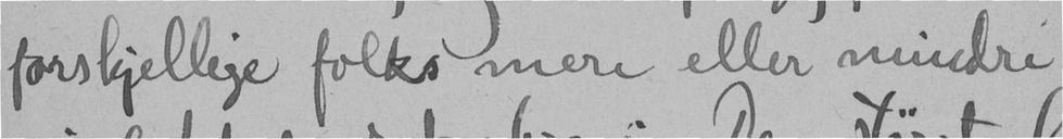forskjellige folks mere eller mindre
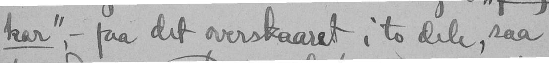kar", - faa det overskaaret i to dele, saa
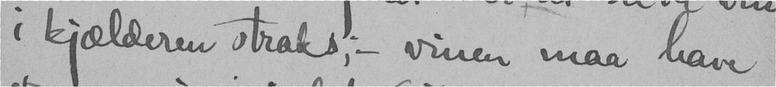i kjælderen straks; - vinen maa have
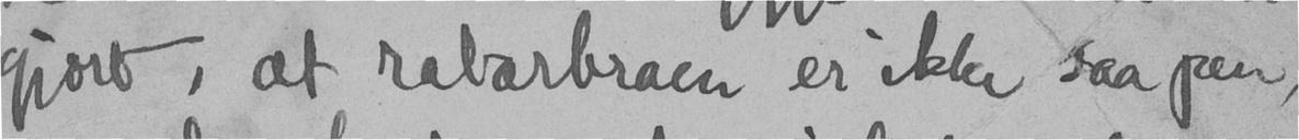gjort, at rabarbraen er ikke saa pen,
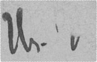Hr.
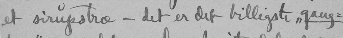et sirupstræ - det er det billigste "gang-
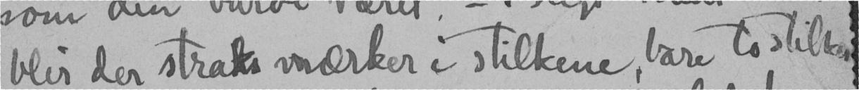blir der straks mærker i stilkene, bare to stilker
